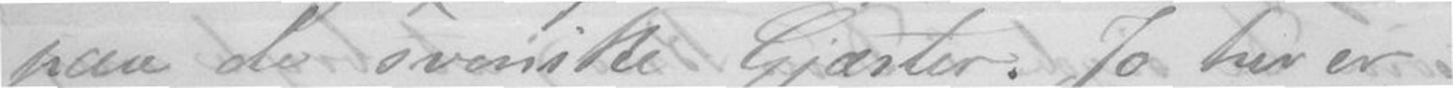paa de svenske Gjæster. Jo, her er
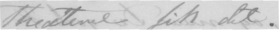Theateret fik det.
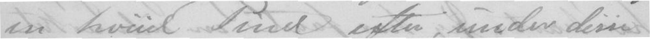en hviid Pind efter, under disse
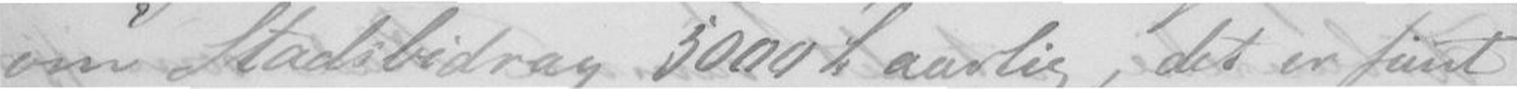om Stadsbidrag 5000 £. aarlig, det er fint
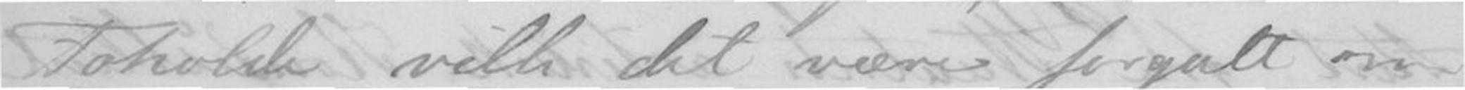Forholde ville det være forgalt om
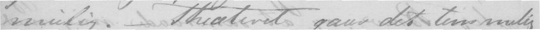mulig. - Theateret gaar det temmelig
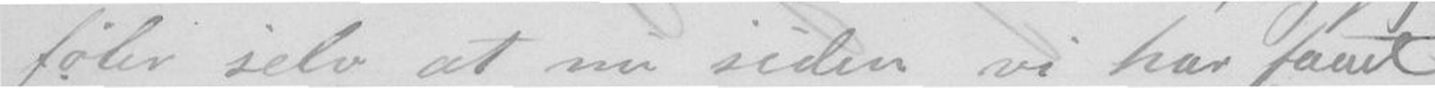føler selv at nu siden vi har faaet
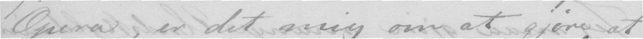Opera, er det mig om at gjøre at
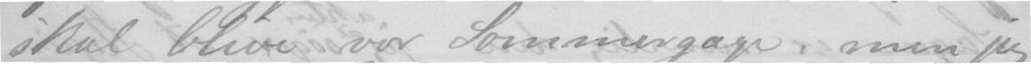skal blive vor Sommergage, men jeg
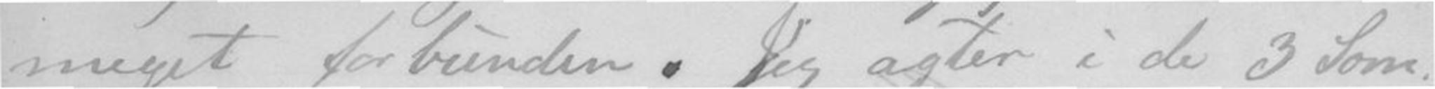meget forbunden. Jeg agter i de 3 Som-
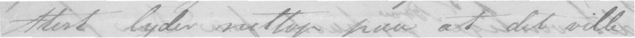Atest lyder nettop paa at det ville
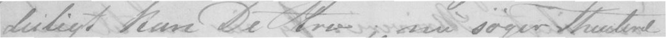deiligt kan De tro, nu søger Theateret
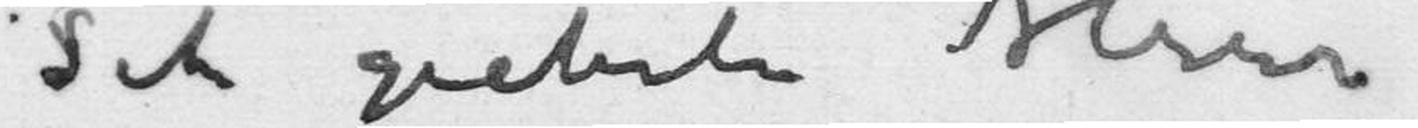Sehr geehrte Hrrn
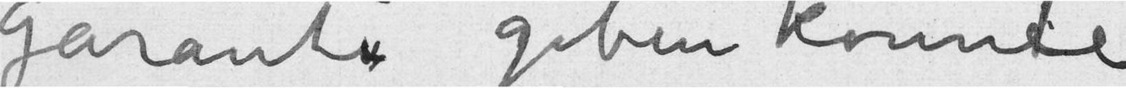Garanti geben könnte
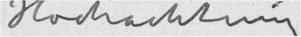Hochachtung
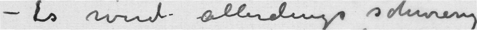– Es werde allerdings schwierig
